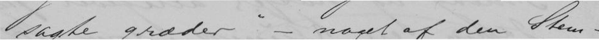sagte græder" - noget af den Stem-
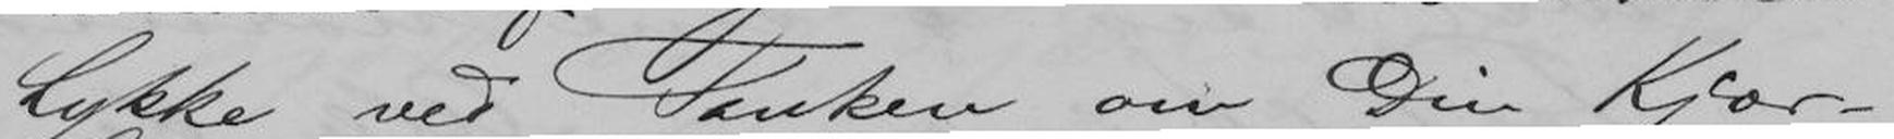Lykke ved Tanken om Din Kjær-
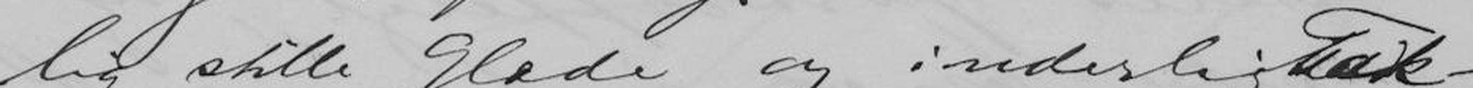lig stille Glæde og inderlig Tak-
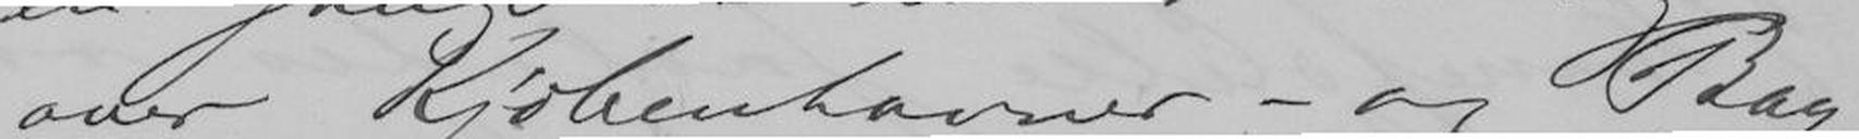over Kjøbenhavner- og Bay-
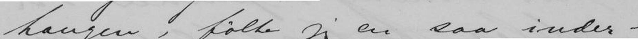haugen, følte jeg en saa inder-
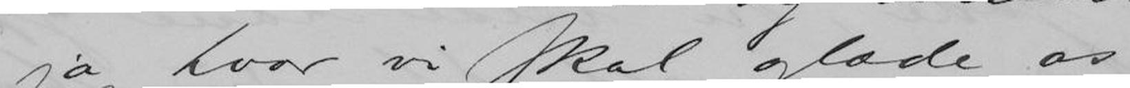ja hvor vi skal glæde os
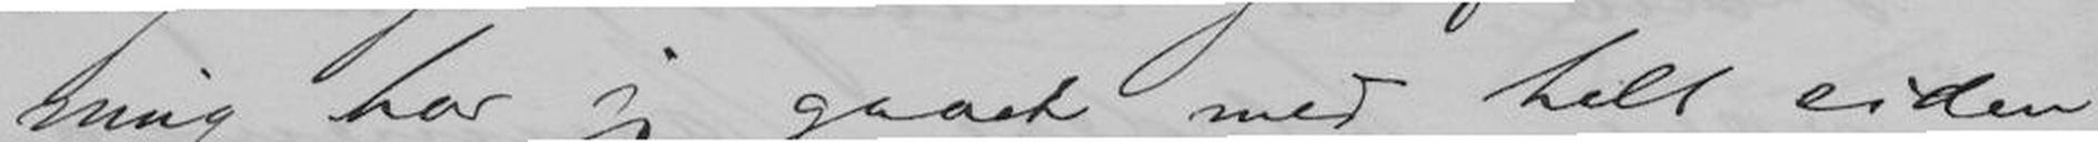ning har jeg gaaet med helt siden
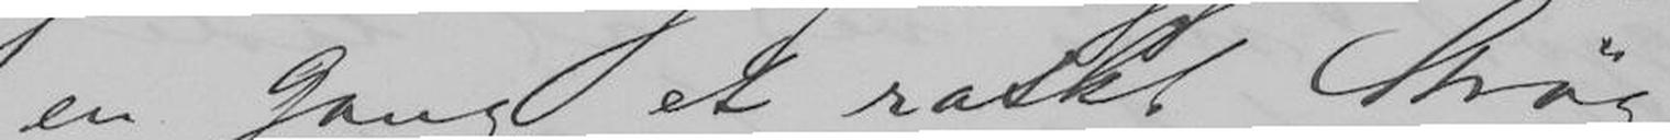en Gang et raskt Strøg
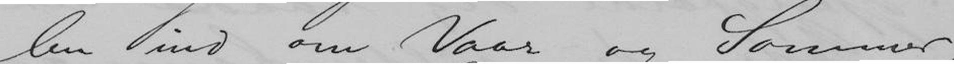len ind om Vaar og Sommer,
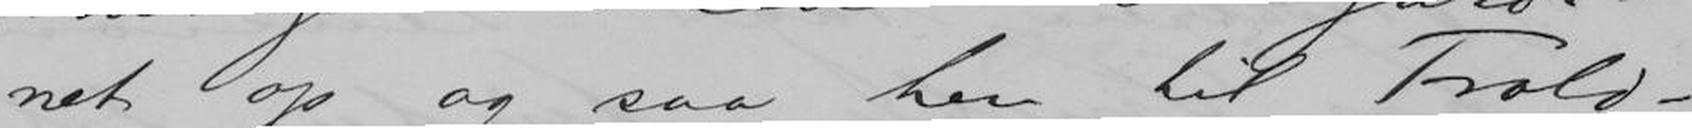net op og saa hen til Trold-
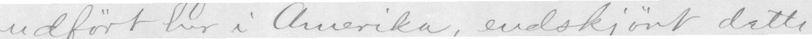udført her i Amerika, endkjønt dette
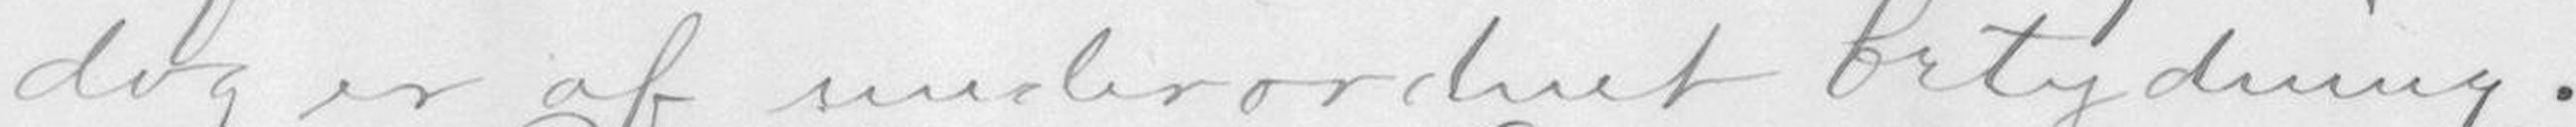dog er af underordnet betydning.
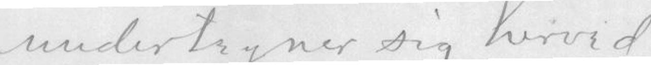undertegner sig herved
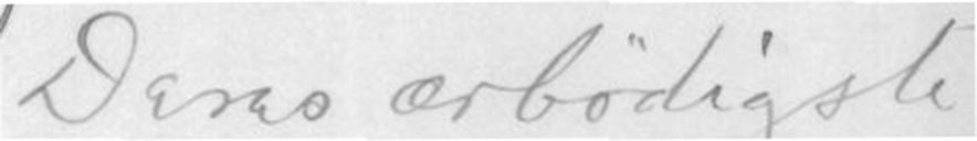Deres ærbødigste
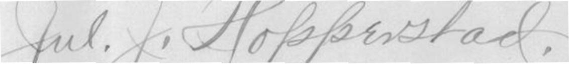Jul Z.Hopperstad
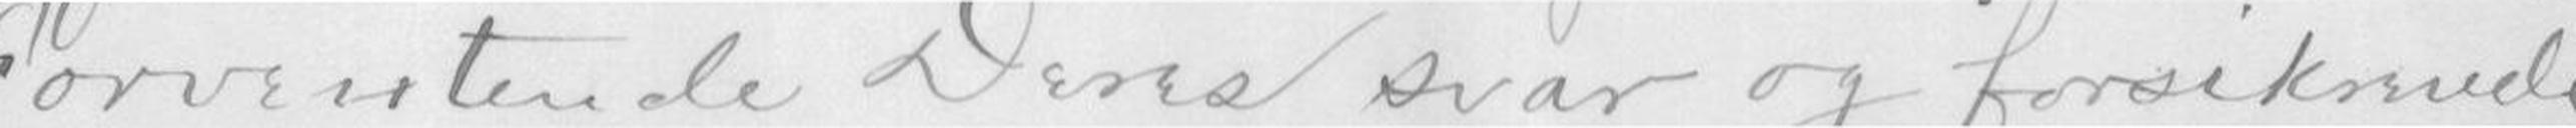Forventende Deres svar og forsikrevde
